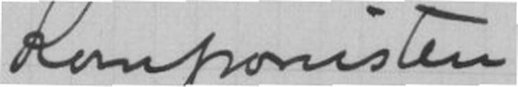Komponisten
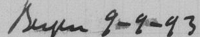Bergen 9-9-93
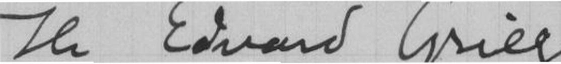Hr. Edvard Grieg
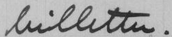billetter.
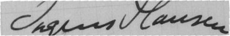Irgens Hansen
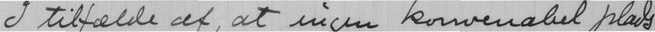I tilfælde af, at ingen konvenabel plads
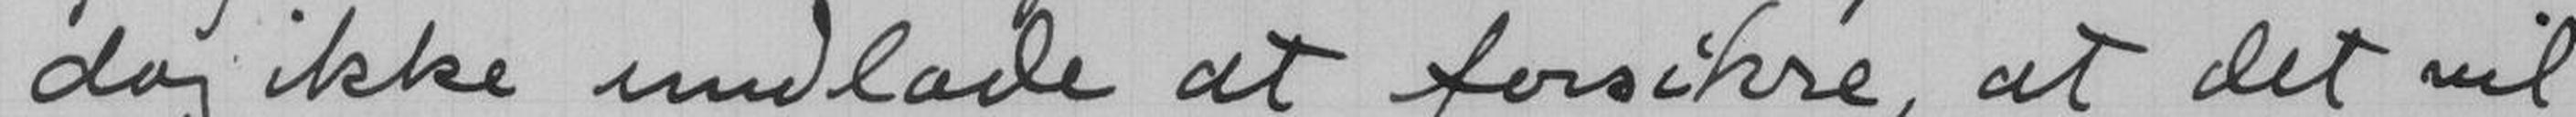dog ikke undlade at forsikre, at det vil
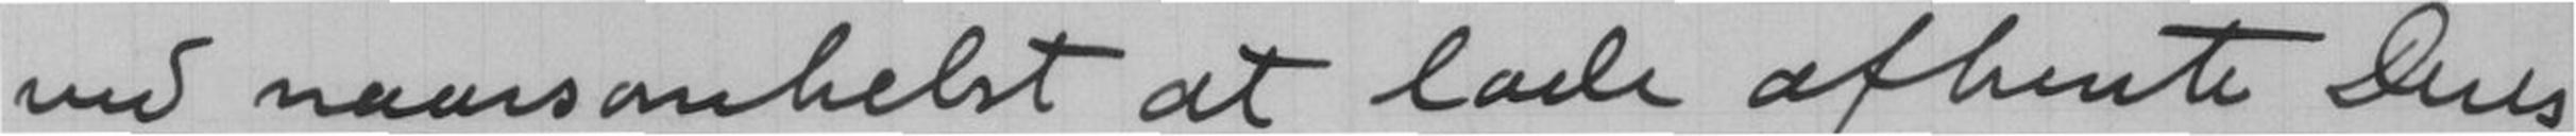ved naarsomhelst at lade afhente Deres
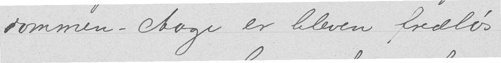dommen. Aage er bleven fredløs
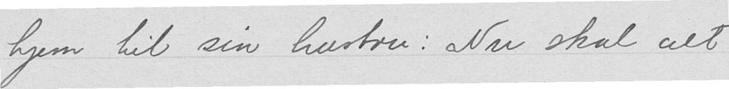hjem til sin hustru: Nu skal alt
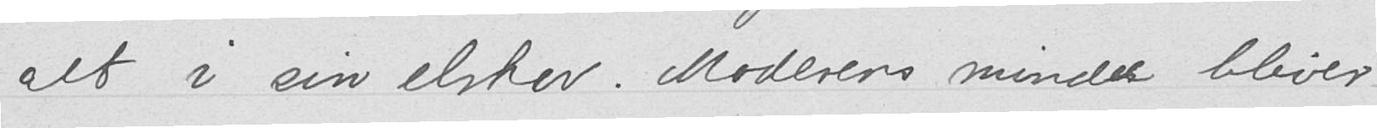alt i sin elskov. Moderens minder bliver
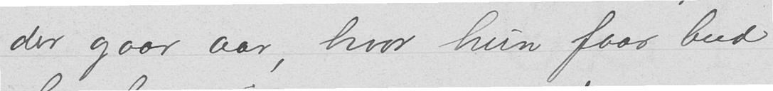der gaar aar, hvor hun faar bud
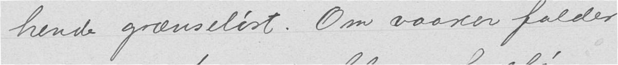hende grænseløst. Om vaaren falder
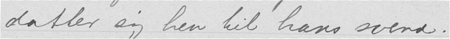datter sig hen til hans svend.
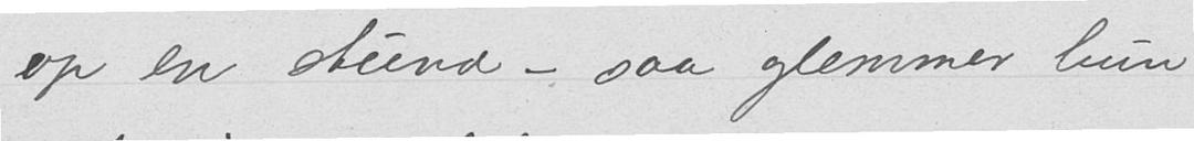op en stund – saa glemmer hun
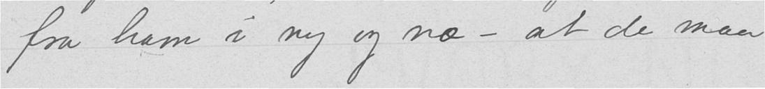fra ham i ny og næ – at de maa
